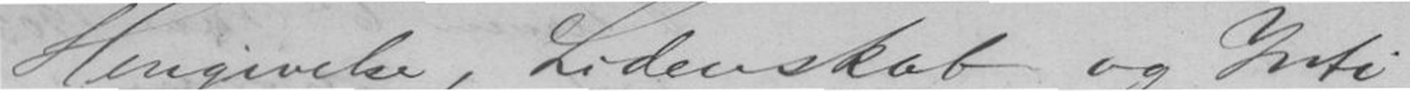Hengivelse, Lidenskab og Inti-
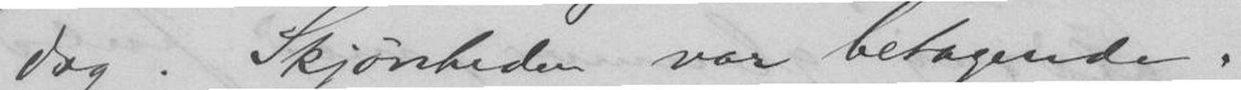dag. Skjønheden var betagende.
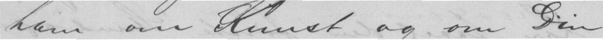ham om Kunst og om Din
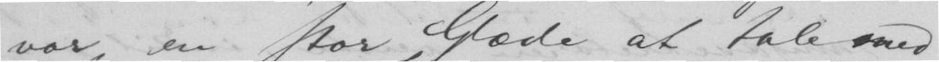var en stor Glæde at tale med
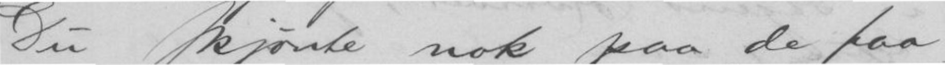Du skjønte nok paa de faa,
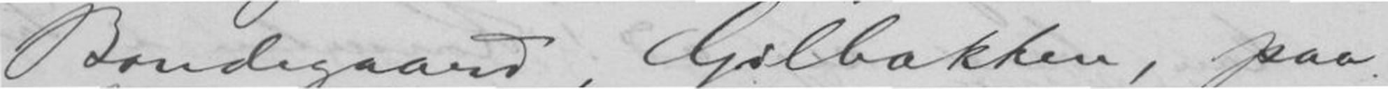Bondegaard, Gilbakken, paa
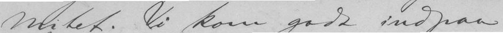mitet. Vi kom godt indpaa
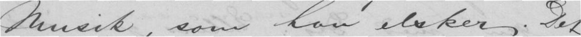Musik, som han elsker. Det
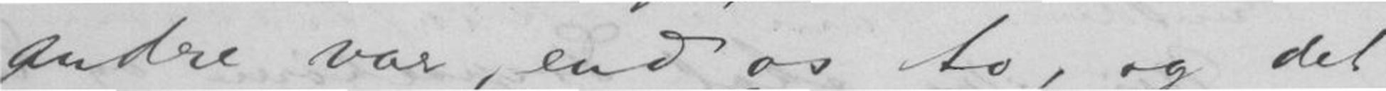andre var, end os to, og det
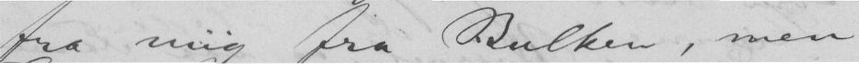hørte fra mig fra Bulken, men
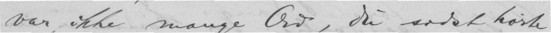var ikke mange Ord, du sidst
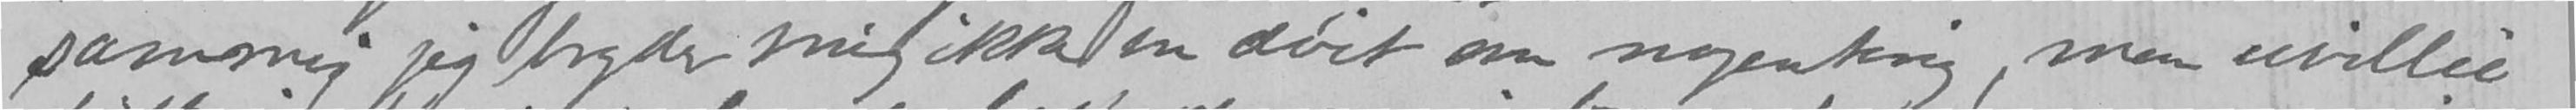samme jeg bryder mig ikke en døit om nogenting, men uvillie
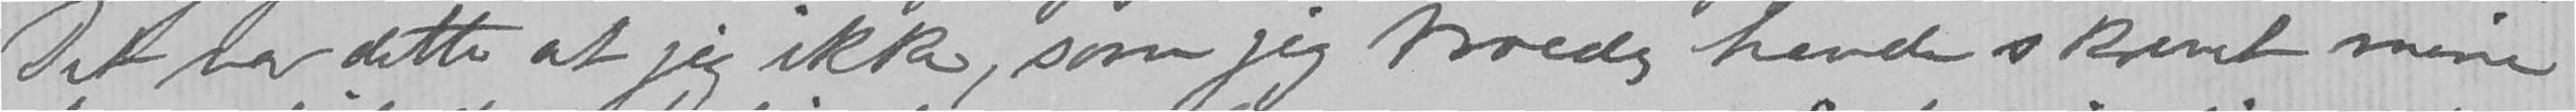Det var dette at jeg ikke, som jeg troede havde skrevet mine
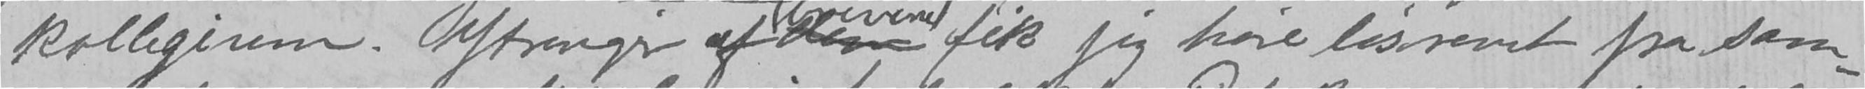kollegium. Ytringen af brevene fik jeg høre løsrevet fra sam-
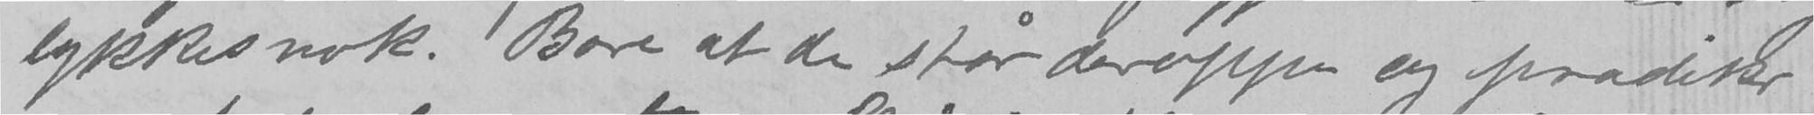lykkes nok. Bare at de står deroppe og prædiker
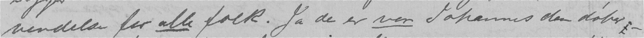vendelse for alle folk. Ja de er vor Johannes den døber.-
